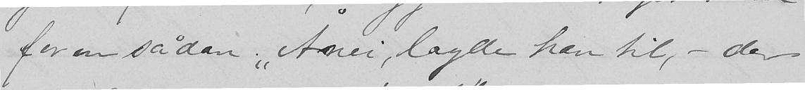for en sådan. "Ånei, lagde han til, - der
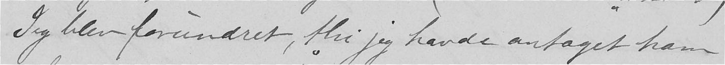Jeg blev forundret, thi jeg havde antaget ham
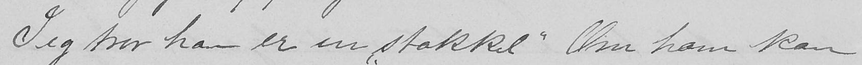Jeg tror han er en "stakkel" Om ham kan
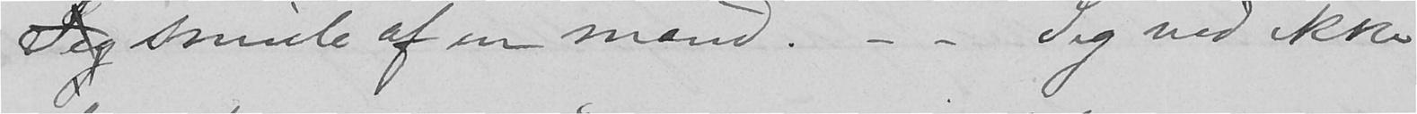jeg smule af en mand. - - Jeg ved ikke
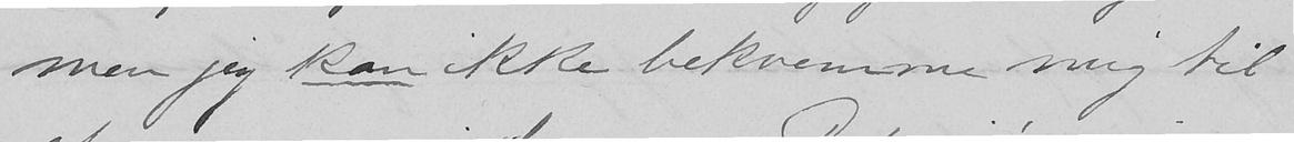men jeg kan ikke bekvemme mig til
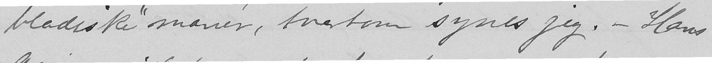bladiske" manér, tvertom synes jeg. - Hans
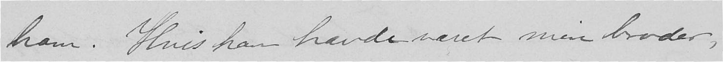ham. Hvis han havde været min broder,
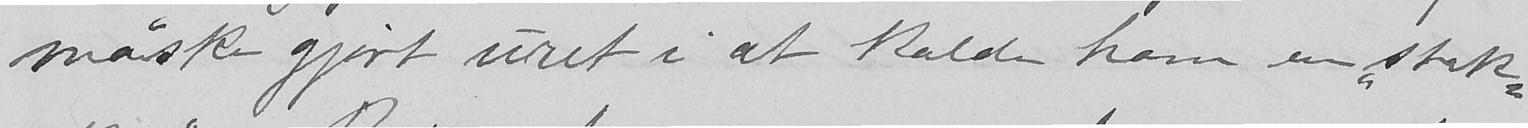måske gjort uret i at kalde ham en "stak-
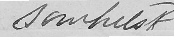somhelst
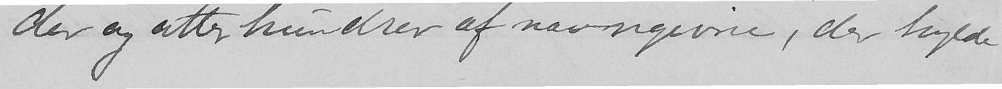der og atter hundrer af navngivne, der hylde
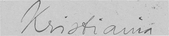Kristiania
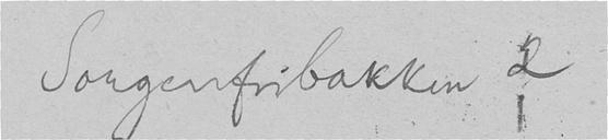Sorgenfribakken 2
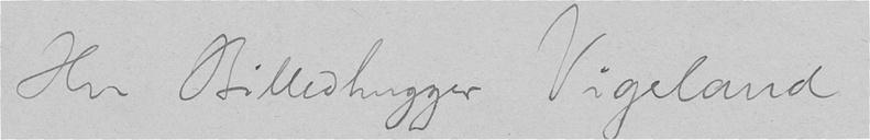Hr Billedhugger Vigeland
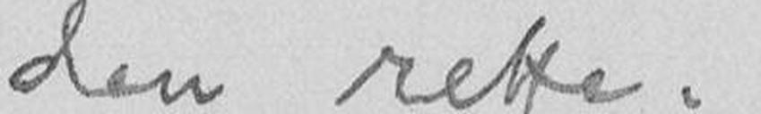den rette.
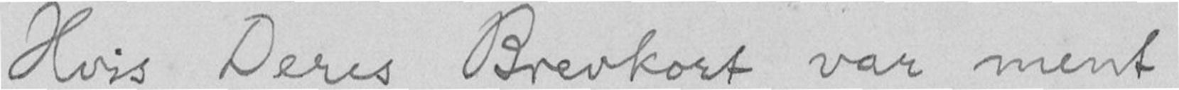Hvis Deres Brevkort var ment
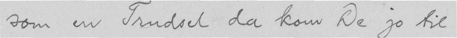som en Trudsel da kom De jo til
